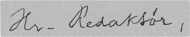Hr. Redaktør,
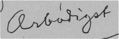Ærbødigst
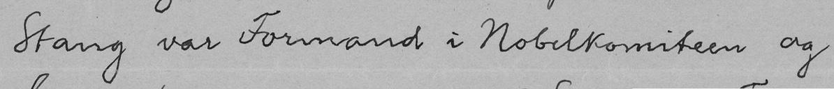Stang var Formand i Nobelkomiteen og
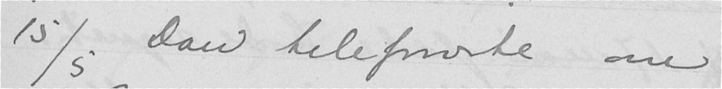15/5 Don telefonerte om
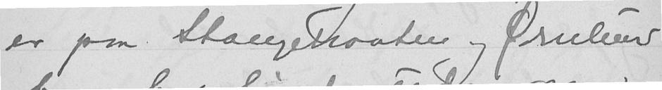er paa Stangebraaten og Ørnlund
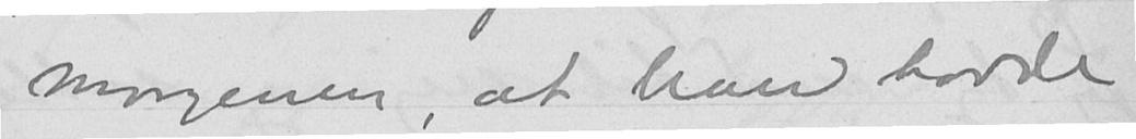morgenen, at han havde
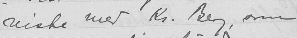reiste med Kr. Berg, som
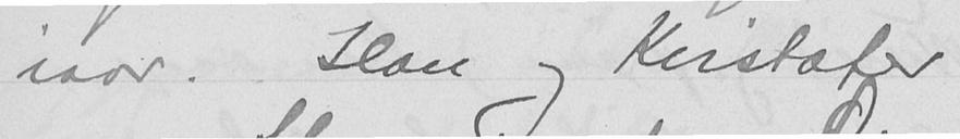iaar. Han og Kristofer
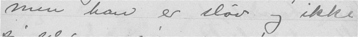men han er sløv og ikke
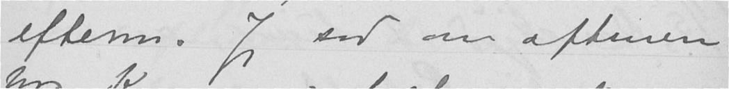efterm. Jeg sad om aftenen
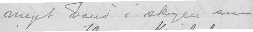meget vand i skogen som
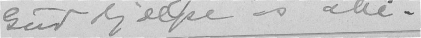Gud hjælpe os alle.
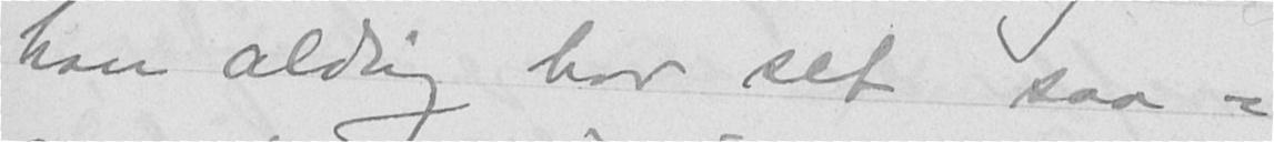han aldrig har set saa-
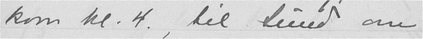kom kl. 4, til Sund om
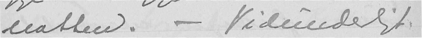natten. - Vidunderligt
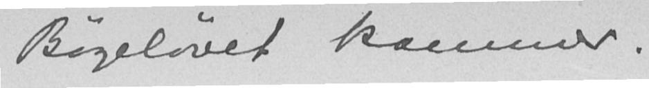Bøgeløvet kommer.
